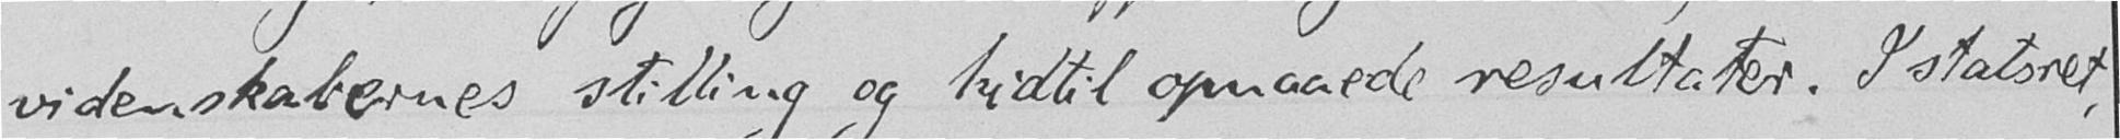videnskabernes stilling og hidtil opnaaede resultater. I statsret,
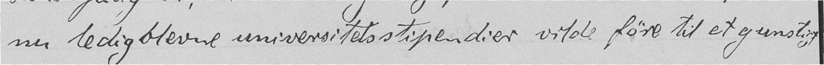nu ledigblevne universitetsstipendier vilde føre til et gunstig
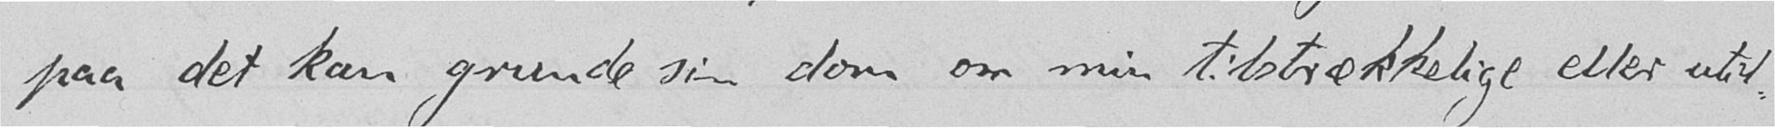paa det kan grunde sin dom om min tilstrækkelige eller util-
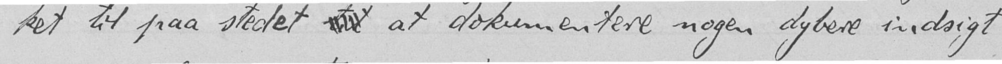ket til paa stedet  at dokumentere nogen dybere indsigt
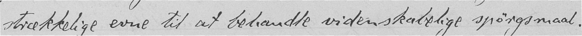strækkelige evne til at behandle videnskabelige spørgsmaal.
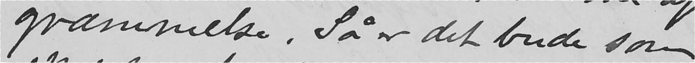græmmelse. Så er det bude som
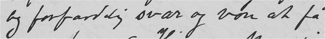og forfærdelig svær og vond at få
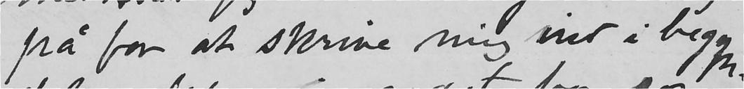på for at skrive mig ind i begyn-
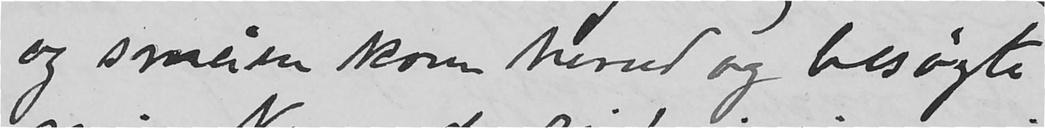og småen kom herned og besøgte
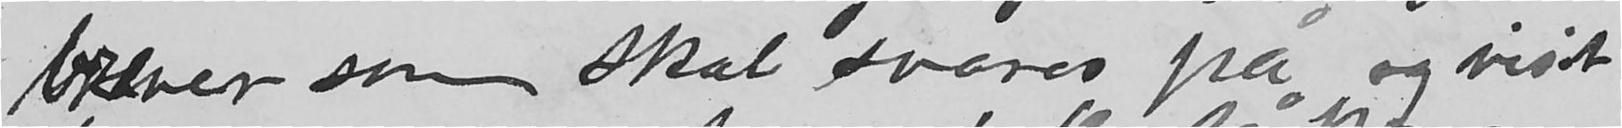brever som skal svares på og visit
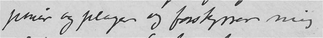piner og plager og forstyrrer mig
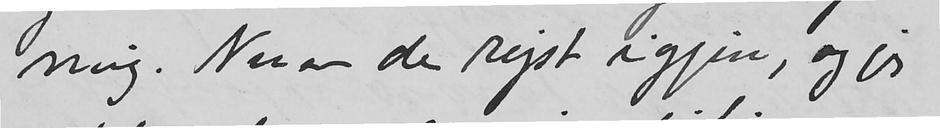mig. Nu er de rejst igjen, og jeg
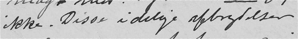ikke. Disse idelige afbrydelser
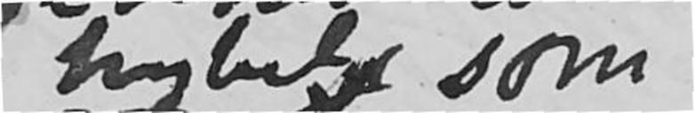hybel som
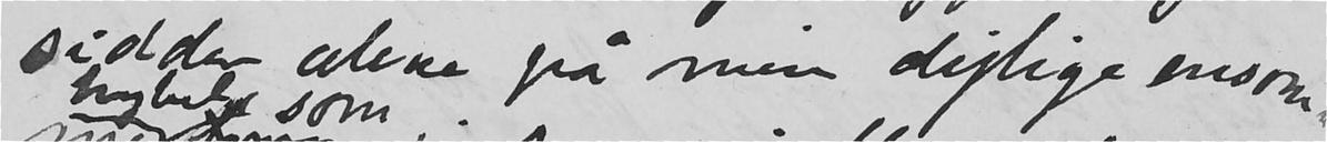sidder alene på min dejlige ensom-
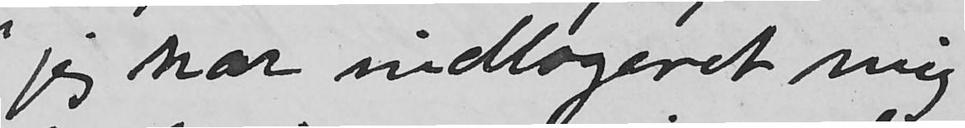jeg har indlogeret mig
